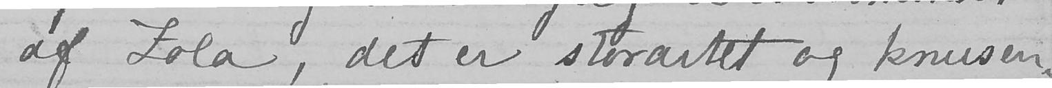af Zola, det er storartet og knusen-
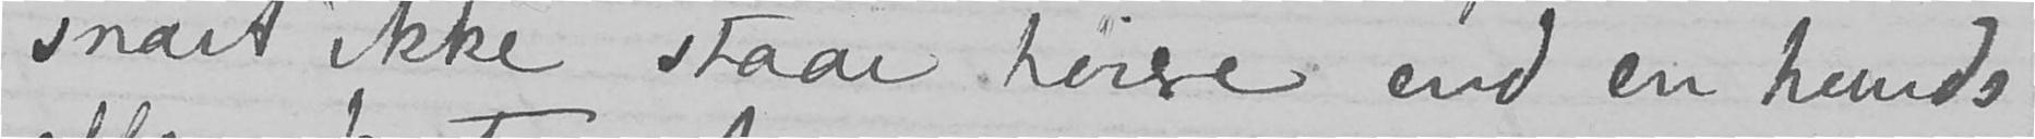snart ikke staar høiere end en hunds
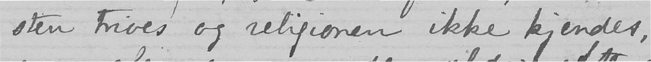sten trives og religionen ikke kjendes,
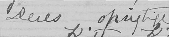Deres oprigtige
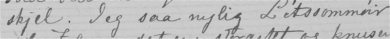skjel. Jeg saa nylig L'Assommoir
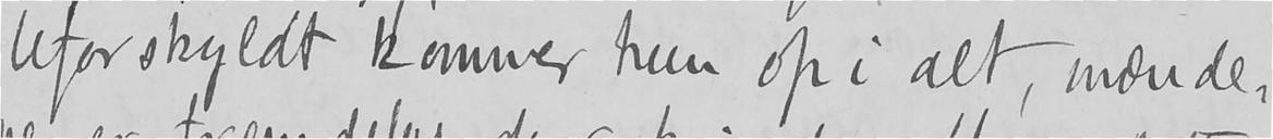Uforskyldt kommer hun op i alt, mænde-
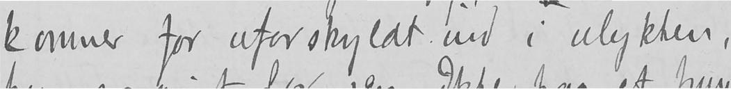kommer for uforskyldt ind i ulykken,
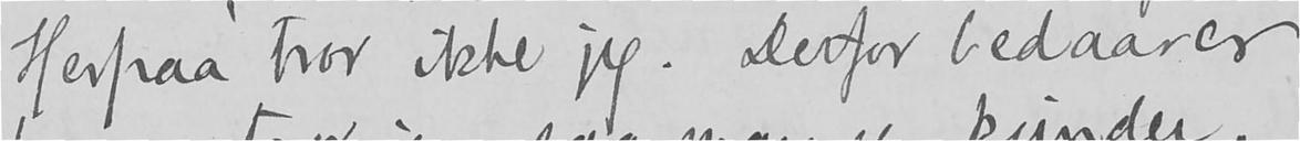Herpaa tror ikke jeg. Derfor bedaarer
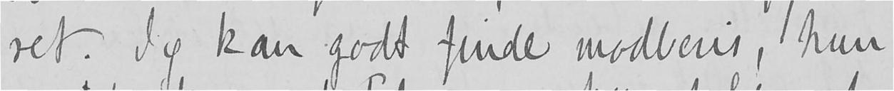ret. Jeg kan godt finde modbevis, hun
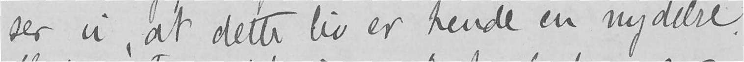ser vi, at dette liv er hende en nydelse.
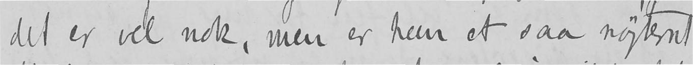det er vel nok, men er hun et saa nøgternt
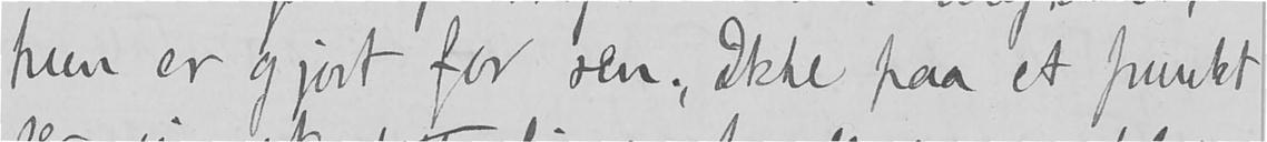hun er gjort for ren. Ikke paa et punkt
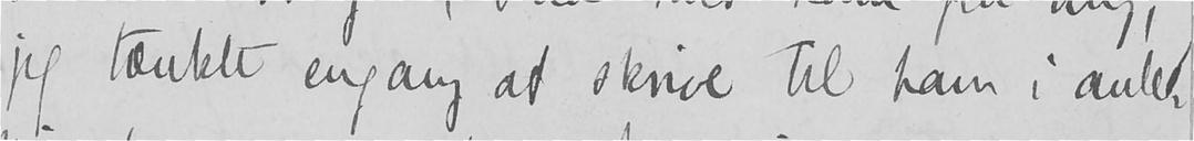jeg tænkte engang at skrive til ham i anled-
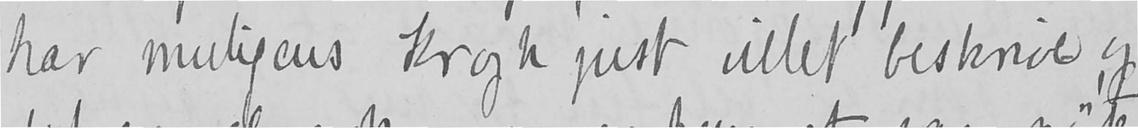har muligens Krogh just villet beskrive, og
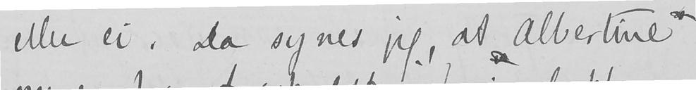eller ei. Da synes jeg, at "Albertine"
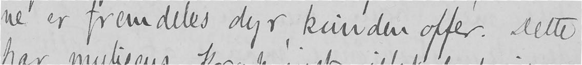ne er fremdeles dyr, kvinden offer. Dette
